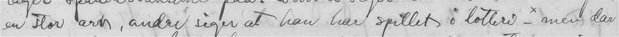en stor arv, andre siger at han har spillet i lotteri - men dar
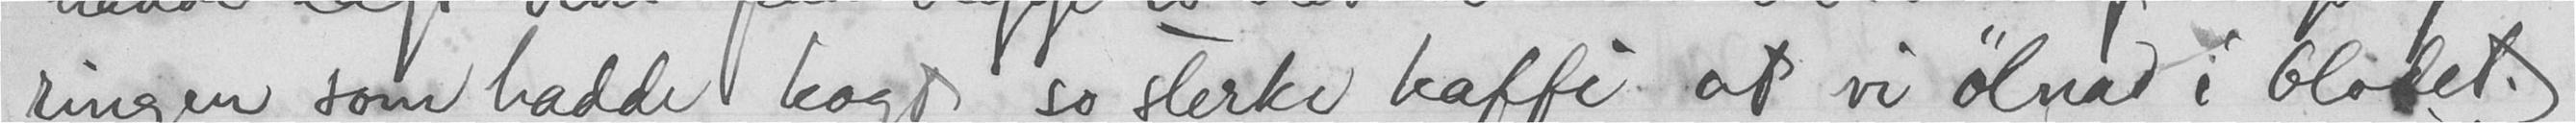ringen som hadde kogt so sterke kaffi at vi ølnad i blodet.
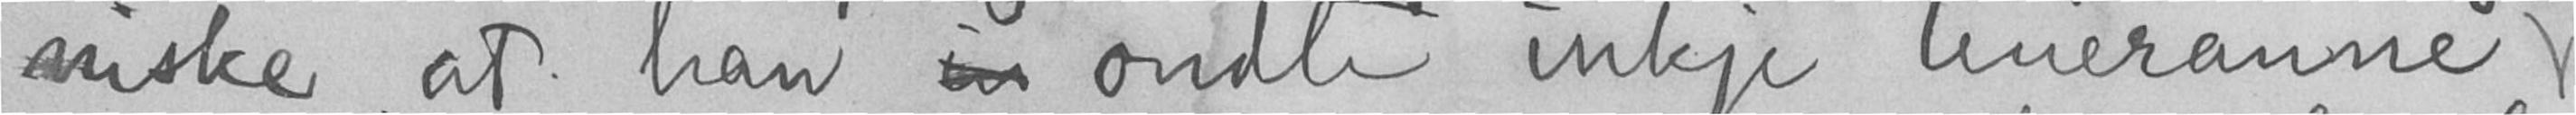niske at han  ondte inkje teneranne
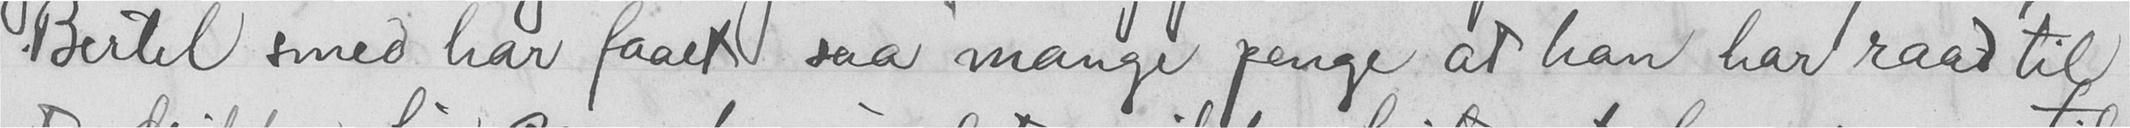Bertel smed har faaet saa mange penge at han har raad til
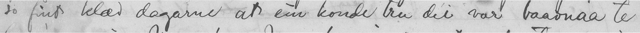so fint klæd dagarne at ein konde tru dei var baadnaa te
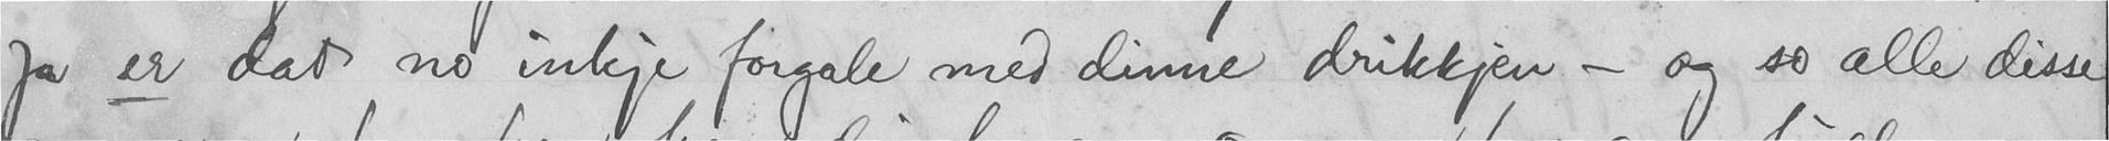Ja er dat no inkje forgale med dinne drikkjen - og so alle disse
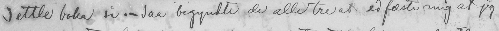ette boka si. - Saa begyndte de alle tre at edfæste mig at jeg
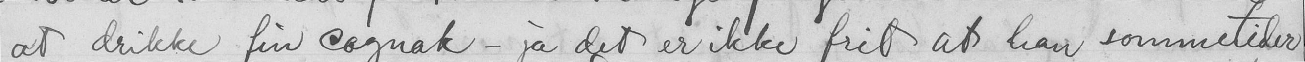at drikke fin cognak - ja det er ikke frit at han sommetider
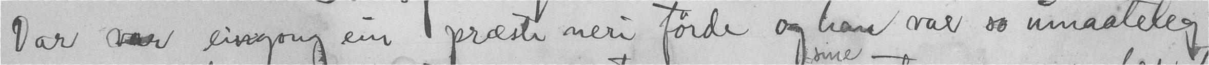Dar var eingong ein præste neri førde og han var so umaateleg
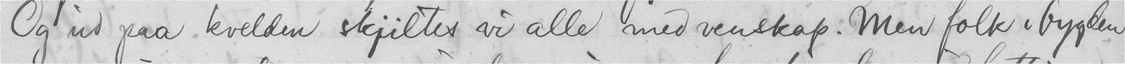Og ud paa kvelden skjiltes vi alle med venskap. Men folk i bygden
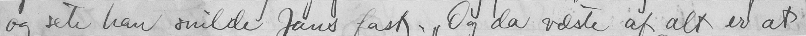og sete han snilde Jans fast. "Og da væste af alt er at
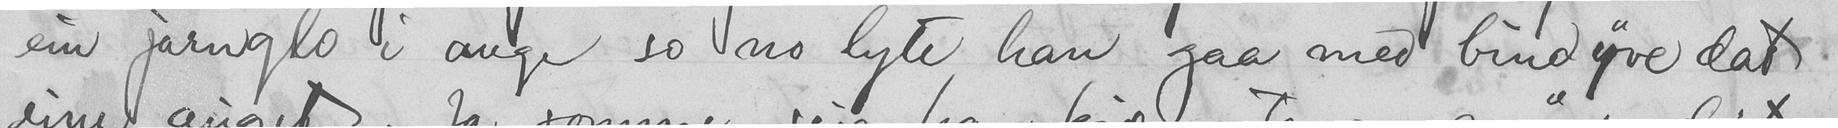ein jarnglo i auge so no lite han gaa med bind yve dat
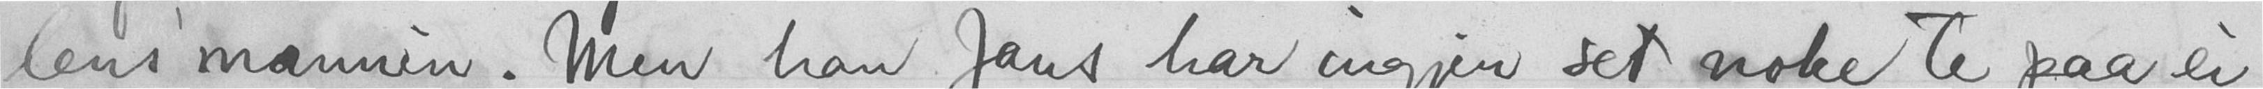lensmannin. Men han Jans har ingjen set noke te paa ei
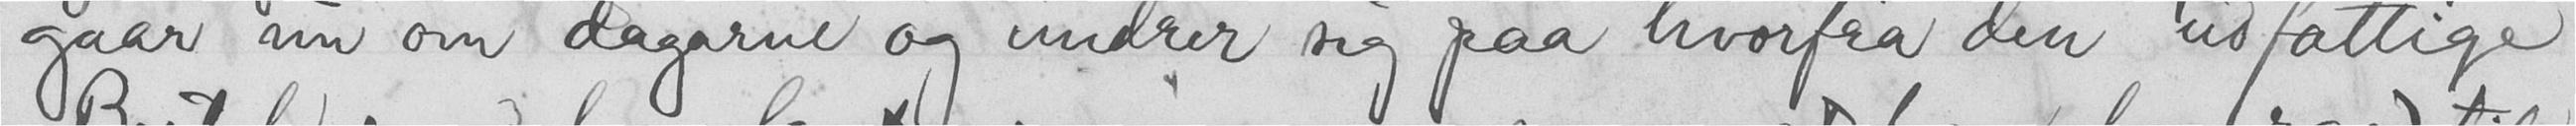gaar nu om dagarne og undrer sig paa hvorfra den udfattige
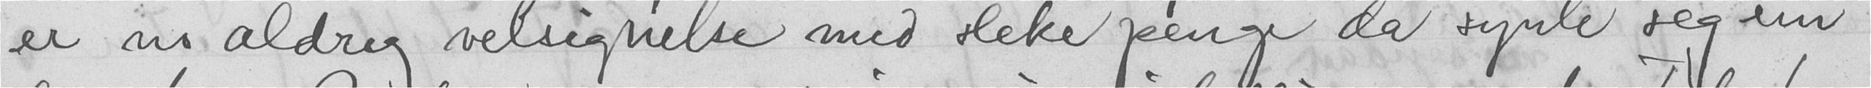er no aldreg velsignelse med sleke penge da synte seg ein
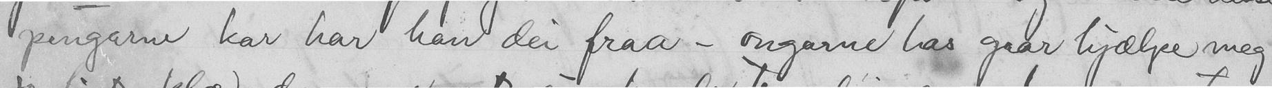pengarne kar har hand dei fraa - ongarne has gaar hjælpe meg
